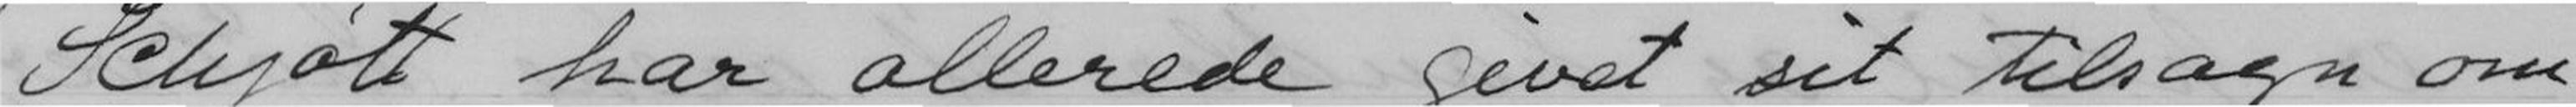Schjøtt har allerede givet sit tilsagn om
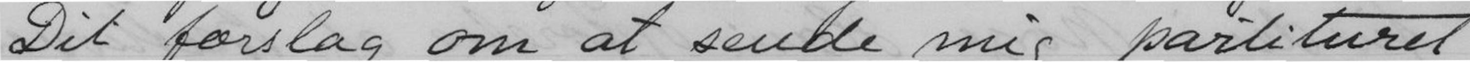Dit forslag om at sende mig partituret
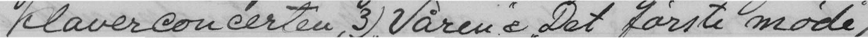klaverconcerten, 3) Våren & Det første møde,
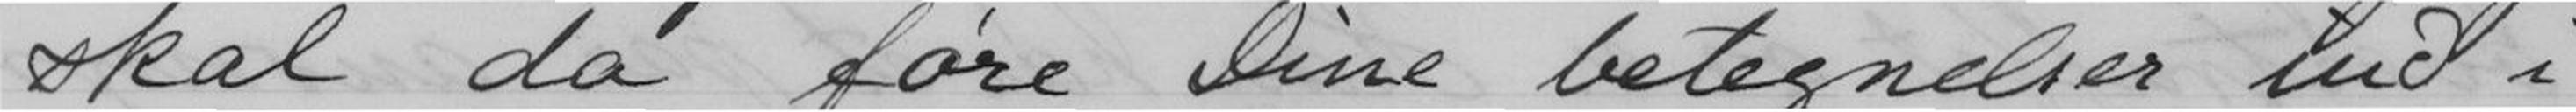skal da føre Dine betegnelser ind i
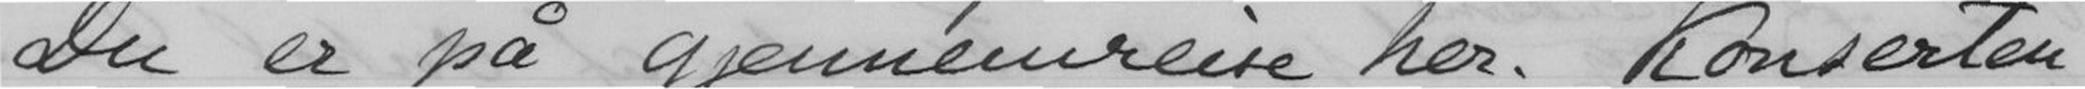Du er på gjennemreise her. Konserten
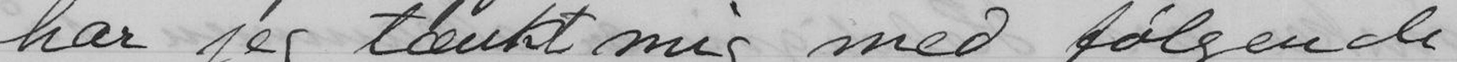har jeg tænkt mig med følgende
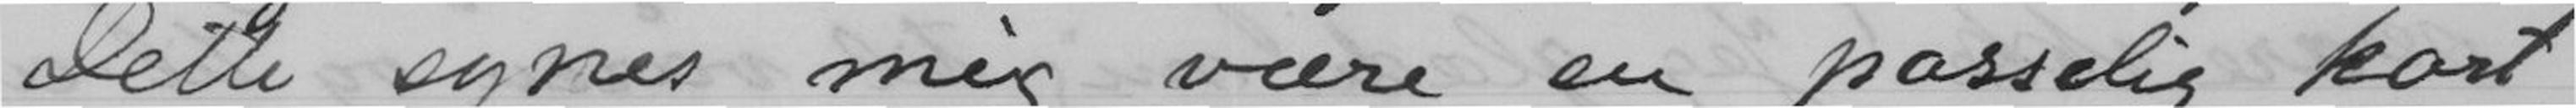Dette synes mig være en passelig kort
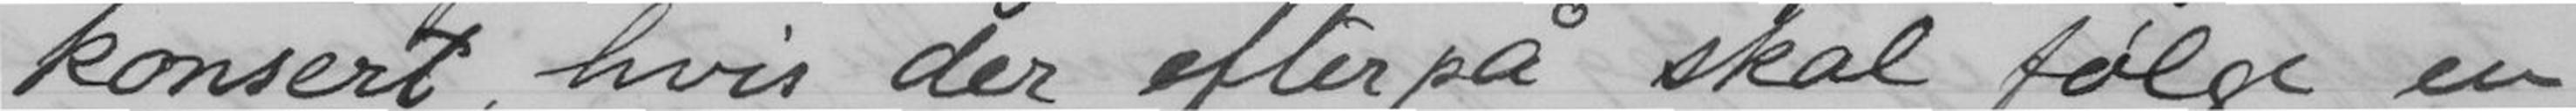konsert, hvis der efterpå skal følge en
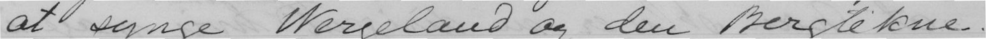at synge Wergeland og den Bergtekne.
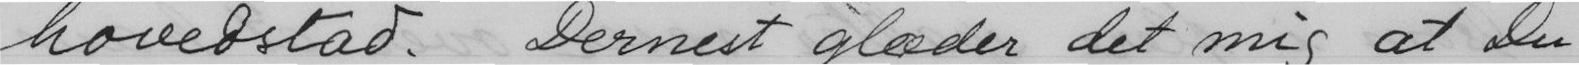hovedstad. Dernest glæder det mig at Du
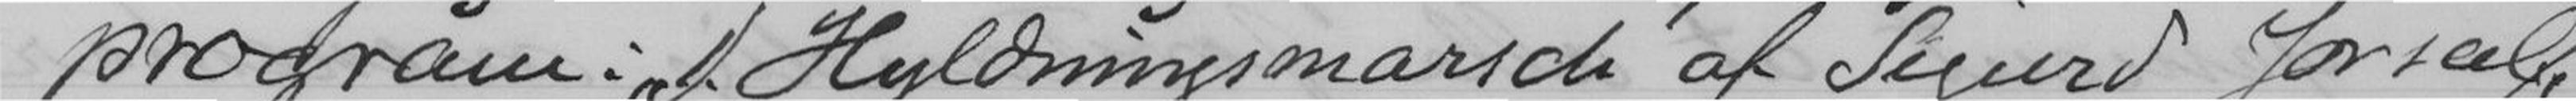program: 1).Hyldningsmarsch af Sigurd Jorsalfar,
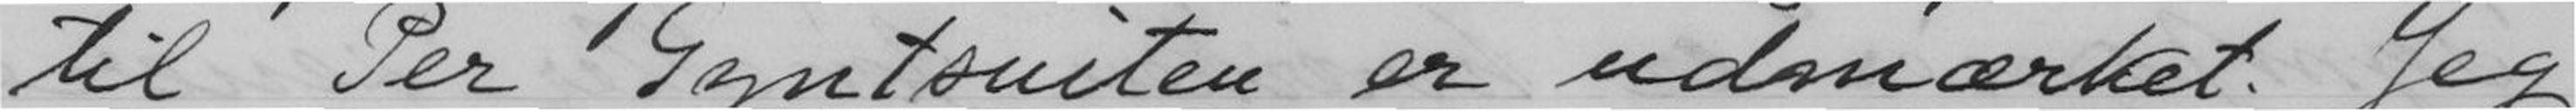til Per Gyntsuiten er udmærket. Jeg
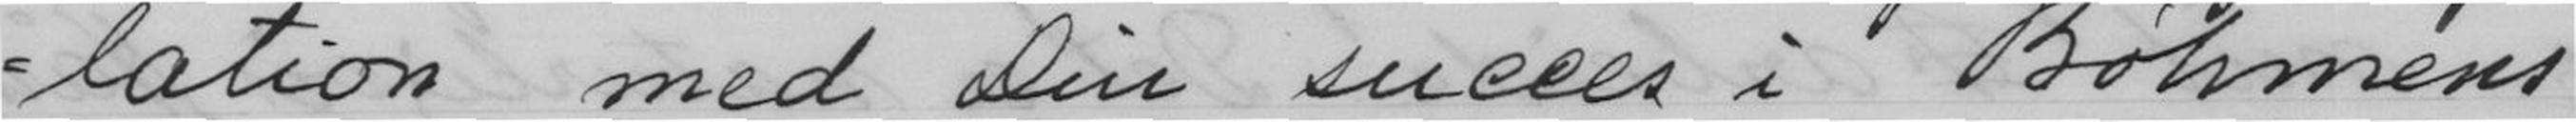lation med Din succes i Bøhmens
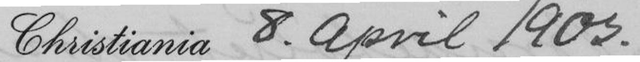Christiania 8. April 1903.
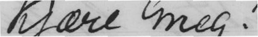Kjære Grieg!
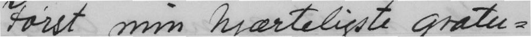Først min hjærteligste gratu-
